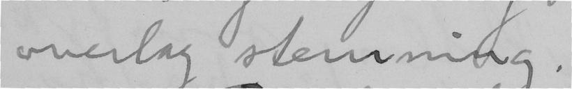overlag stemning.
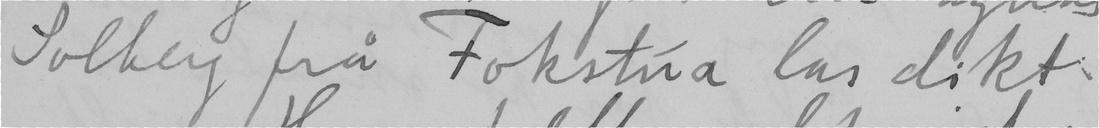Solberg frå Fokstua las dikt.
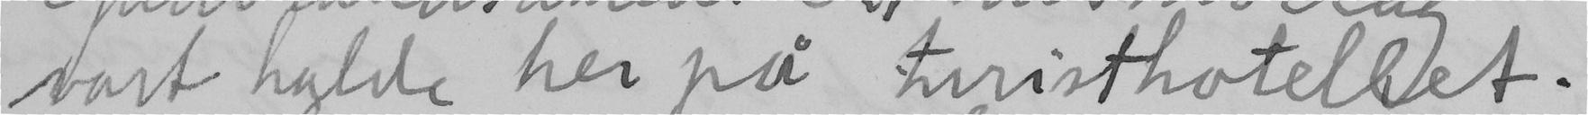vart halde her på turisthotellet.
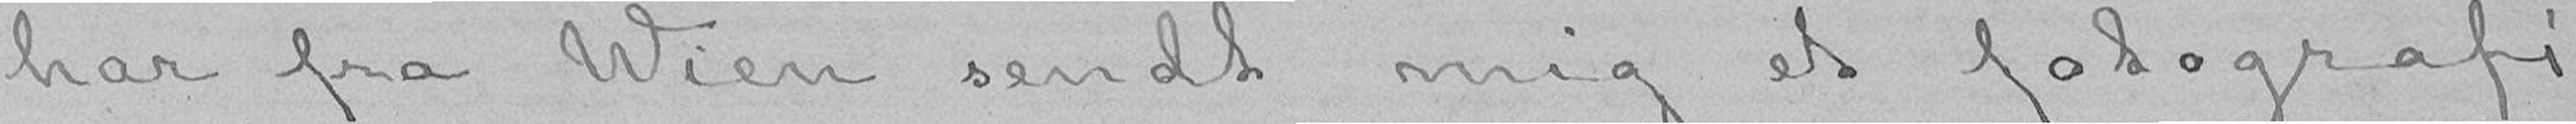har fra Wien sendt mig et fotografi,
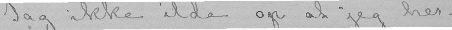Tag ikke ilde op at jeg her-
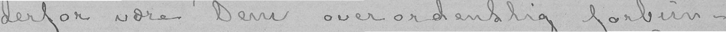derfor være Dem overordentlig forbun-
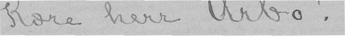Kære herr Arbo!
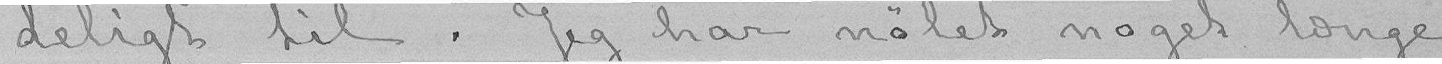deligt til. Jeg har nølet noget længe
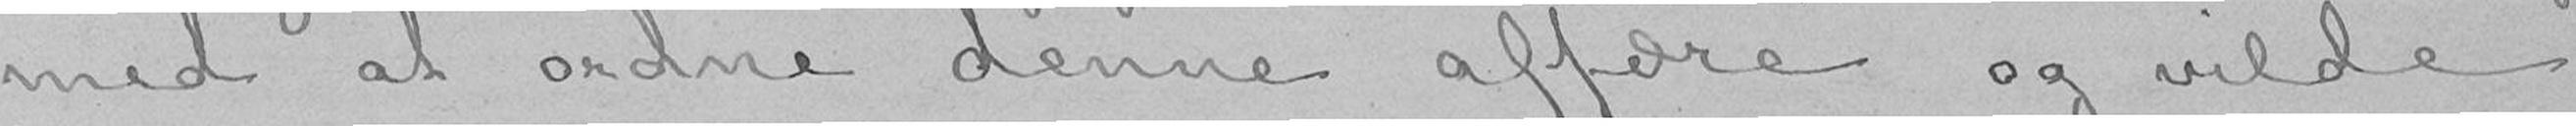med at ordne denne affære og vilde
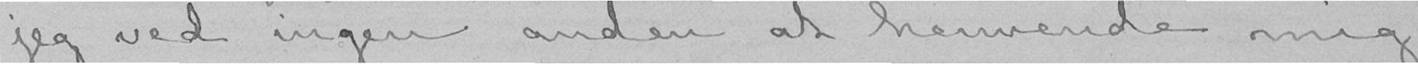jeg ved ingen anden at henvende mig
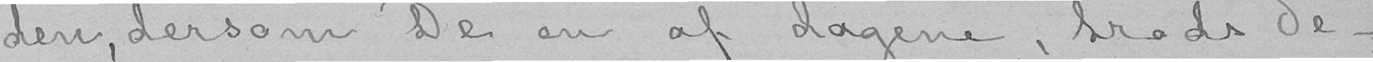den, dersom De en af dagene, trods De-
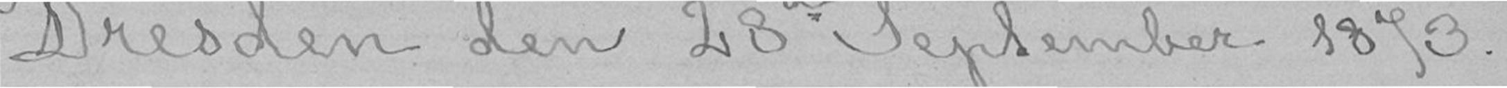Dresden den 28de September 1873.
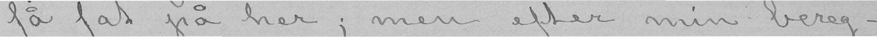få fat på her; men efter min bereg-
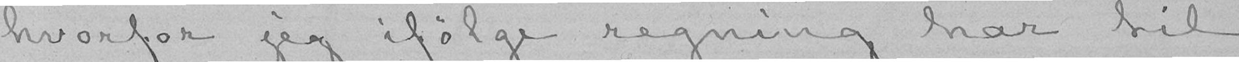hvorfor jeg ifølge regning har til
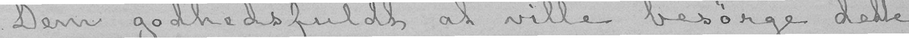Dem godhedsfuldt at ville besørge dette
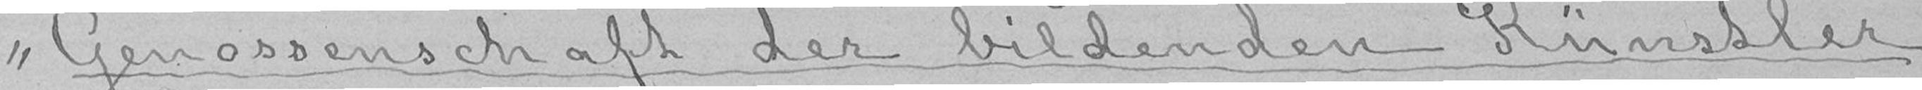«Genossenschaft der bildenden Künstler
